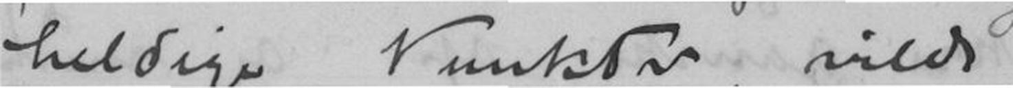heldige Punkter, vilde
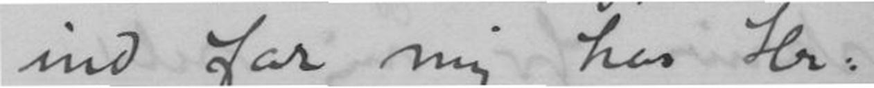ind for mig hos Hr-
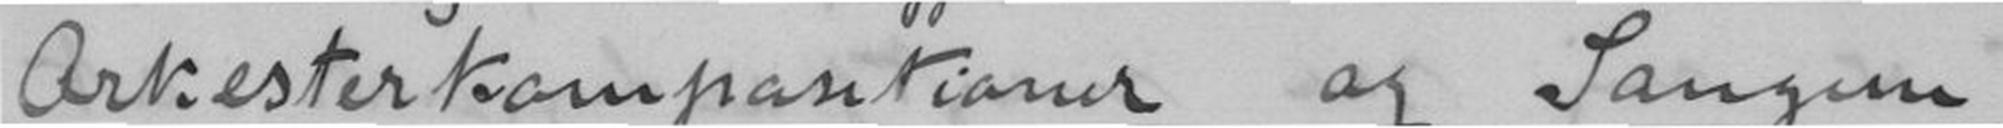Orkesterkompositioner og Sangene,
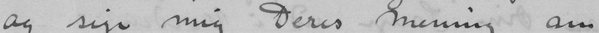og sige mig Deres Menning om
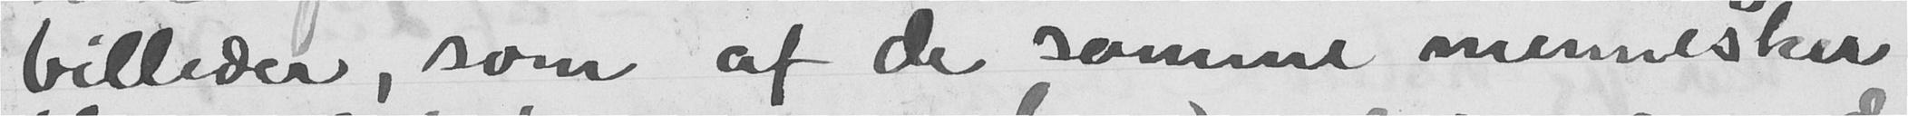billeder, som af de samme mennesker
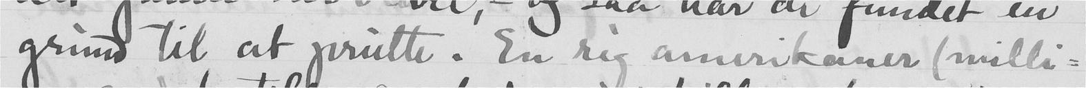grund til at prutte. En rig amerikaner (milli-
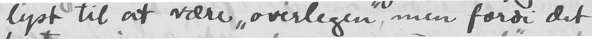lyst til at være "overlegen", men fordi det
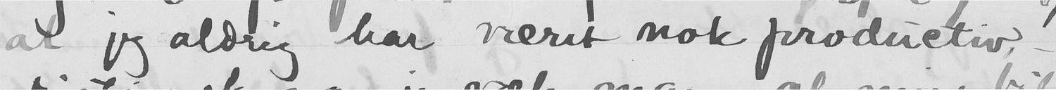at jeg aldrig har været nok productiv, -
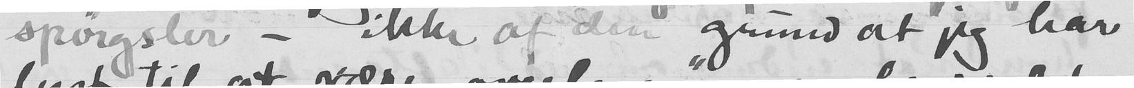spørgsler - ikke af den grund at jeg har
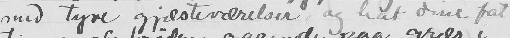med tyve gjæsteværelser, og hat dine fat-
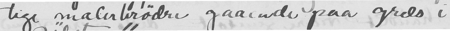tige malerbrødre gaaende paa græs i
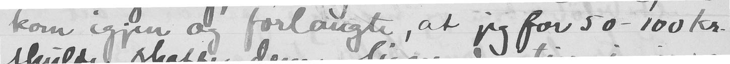kom igjen og forlangte, at jeg for 50 - 100 Kr.
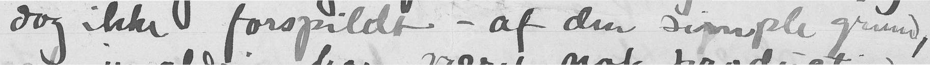dog ikke forspildt - af den simple grund,
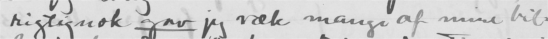rigtignok gav jeg væk mange af mine bil-
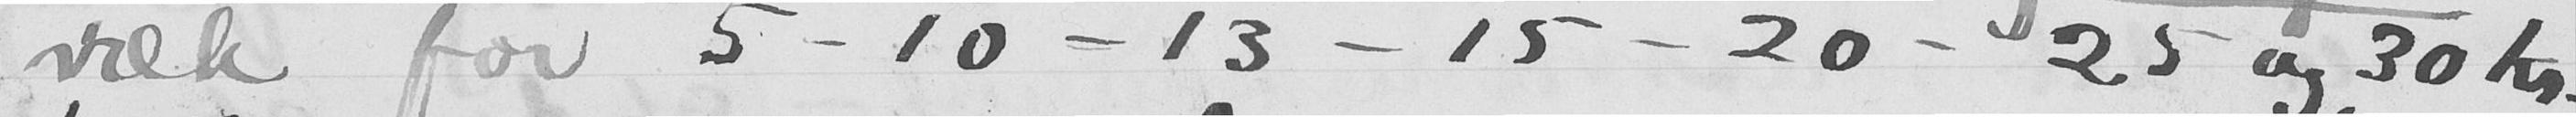væk for 5 - 10 - 13 - 15 - 20 - 25 og 30 kr.
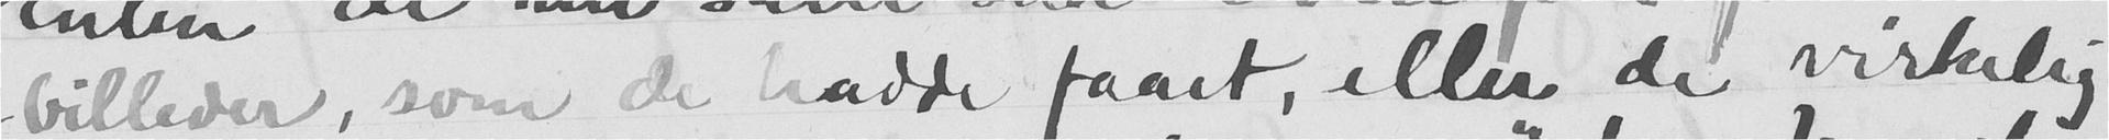billeder, som de hadde faaet, ellar de virkelig
